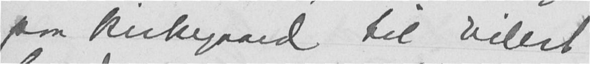paa kirkegaard til Eilert
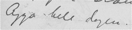Agga hele dagen.
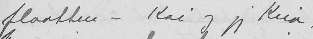flaatten - Kai og jeg Kria,
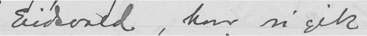Eidsvold, hvor vi gik
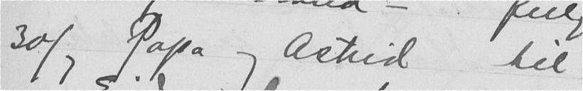30/7 Papa og Astrid til
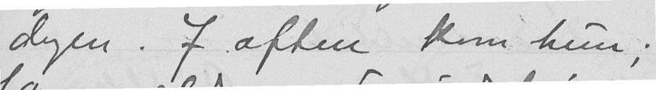dagen. I aften kom hun;
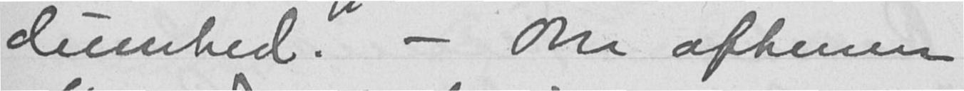dumhed. - Om aftenen
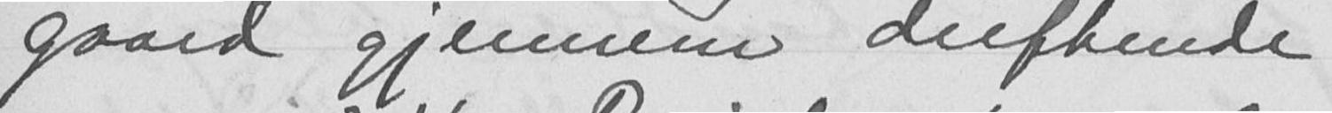gaard gjennem duftende
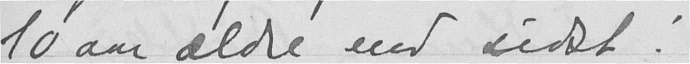10 aar ældre end sidst!
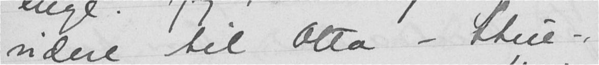videre til Otta - Stue-
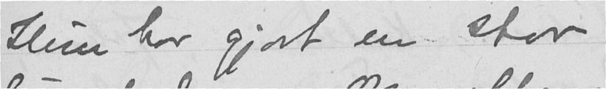Hun har gjort en stor
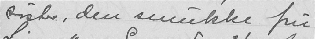søster, den smukke fru
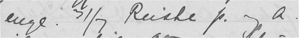enge. 31/7 Reiste p. og A.
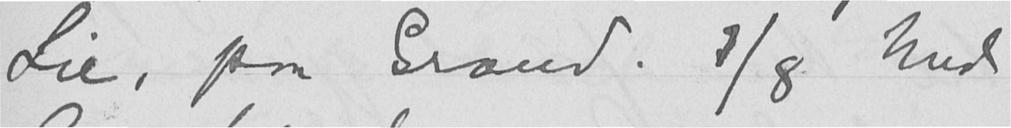Lie, paa Grand. 1/8 Med
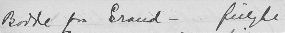Bodde paa Grand - fulgte
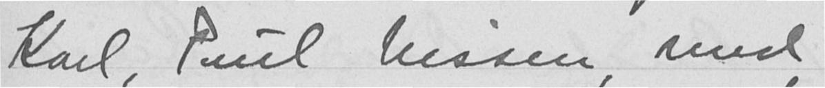Karl, Paul Nissen, med
